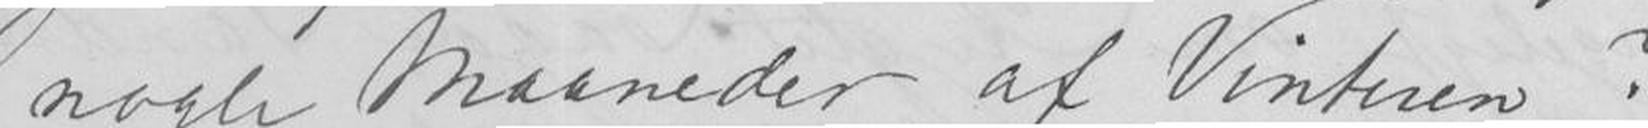nogle Maaneder af Vinteren?
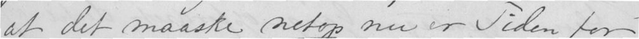at det maaske netop nu er Tiden for
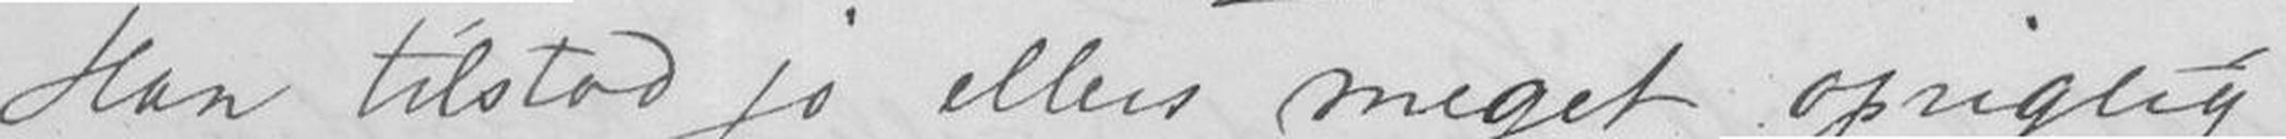Han tilstod jo ellers meget oprigtig
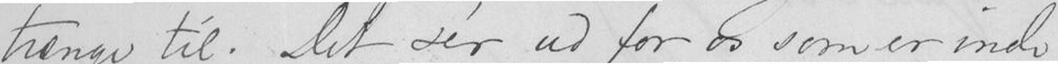trænge til. Det ser ud for os, som er inde
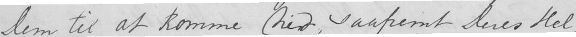Dem til at komme hid, saafremt Deres Hel-
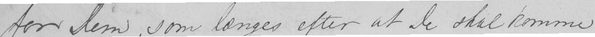for Dem, som længes efter st De skal komme
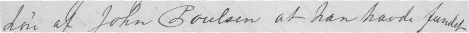høre af John Poulsen at han havde fundet
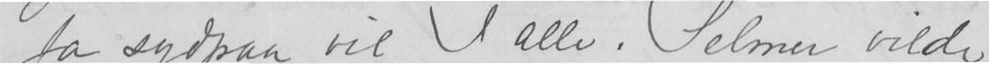Ja sydpaa vil I alle. Selmer vilde
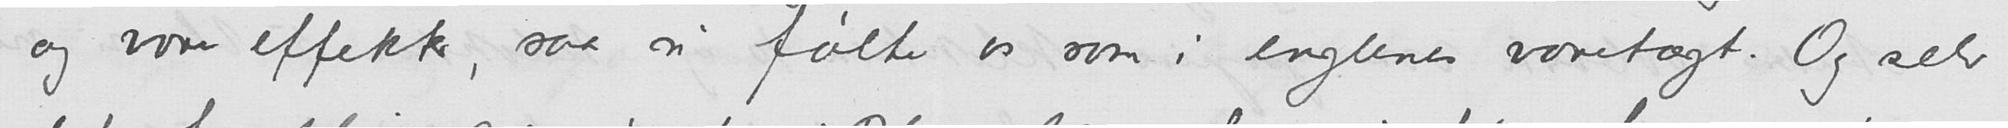og vore effekte, saa vi følte os som i englenes varetægt. Og selv
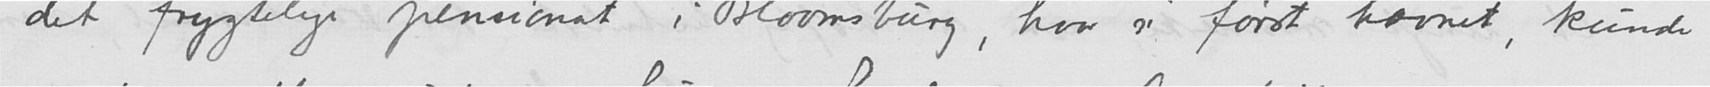det frygtelige pensionat i Bloomsbury, hvor vi først havnet, kunde
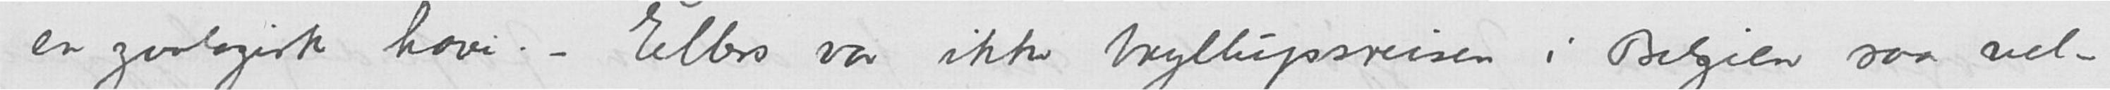en zoologisk have. - Ellers var ikke bryllupsreisen til Belgien saa vel-
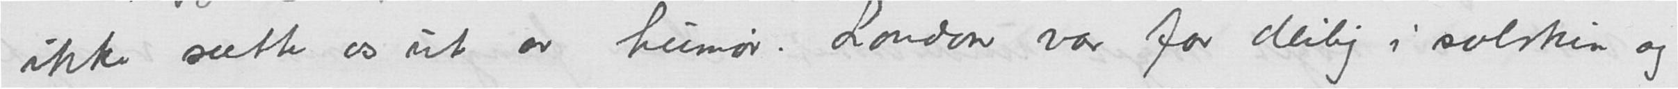ikke sætte os ut av humør. London var for deilig i solskin og
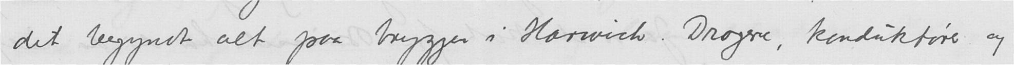det begyndte alt paa bryggen i Harwich. Drogere, konduktører og
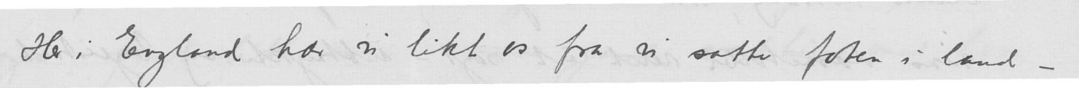Her i England har vi likt os fra vi satte foten i land -
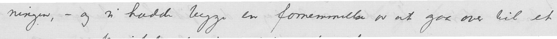ningen, - og vi hadde begge en fornemmelse av at gaa over til et
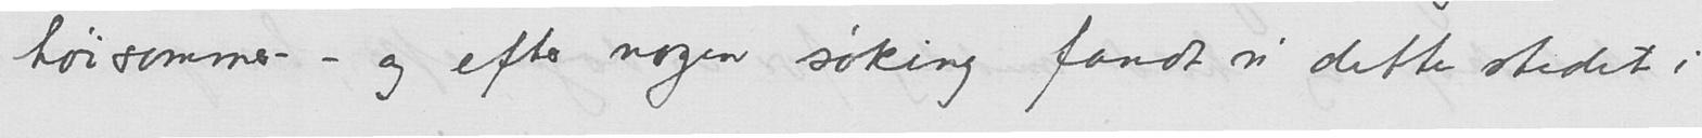høisommer - og efter nogen søking fandt vi dette stedet i
